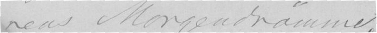rene Morgendrømme,
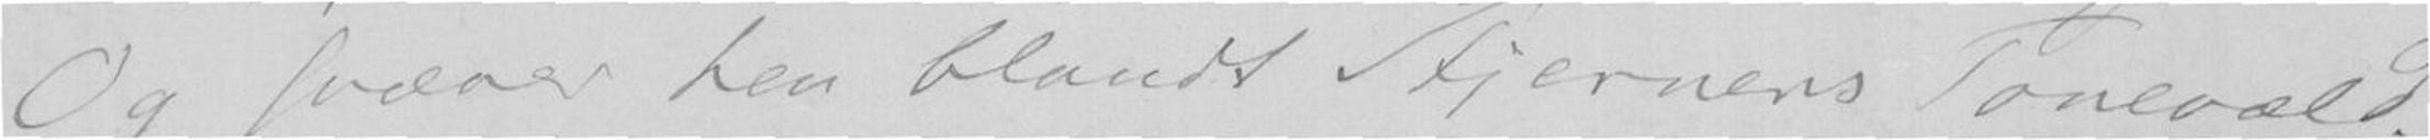Og svæver hen blandt Stjernenes Tonevæld.
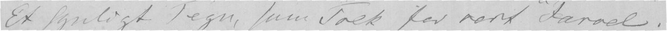Et synligt Tegn, som Tolk for vort "Farvel".
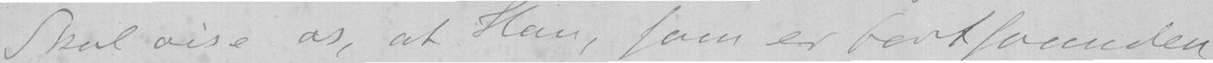Skal vise os, at Han, som er bortsvunden
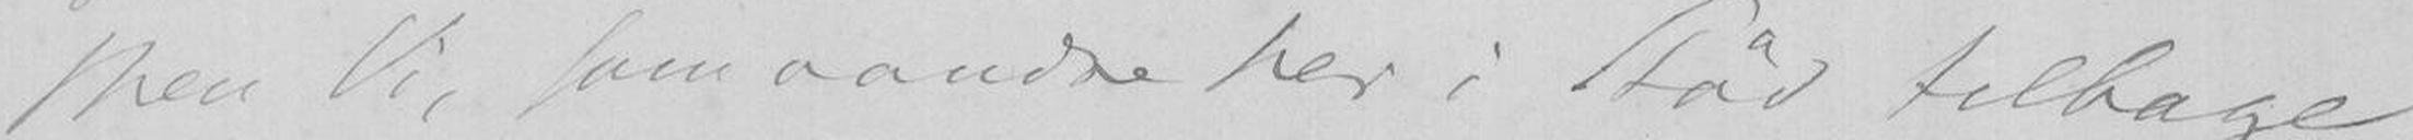Men Vi, som vandre her i Støv tilbage
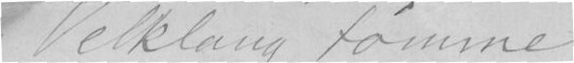Velklang tømme
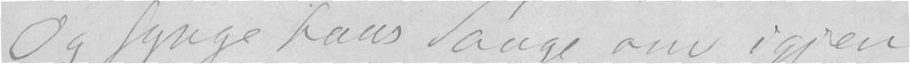Og synge hans Sange om igjen
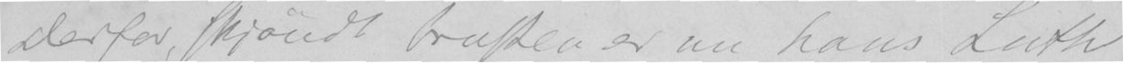Derfor, skjøndt brusten er nu hans Luth,
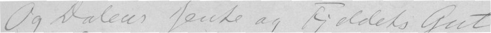Og Dalens Jente og Fjeldets Gut
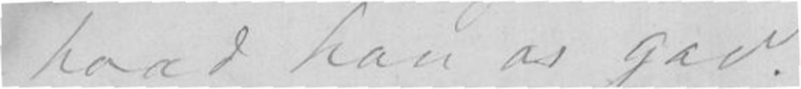hvad han os gav.
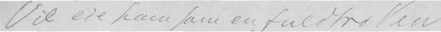Vil eie ham som en fuldtro Ven
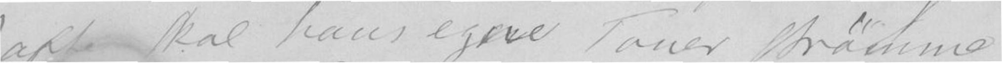I ofte skal hans egne Toner strømme
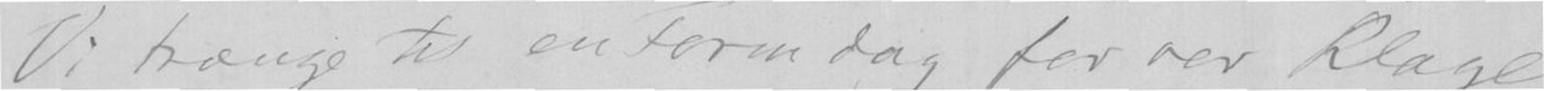Vi trænge til en Form dag for vor Klage
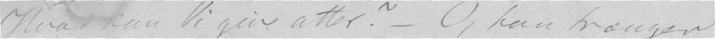Hvad kan Vi give atter? - Og hva trænger
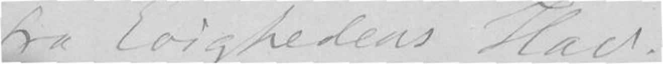fra Evighedens Hav.
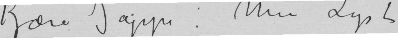Kjære Jappe! Min Lyst
